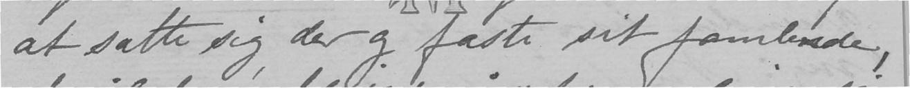at sætte sig der og fæste sit famlende,
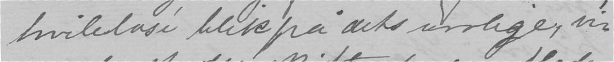hvileløse blik på dets urolige, vi-
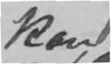kan
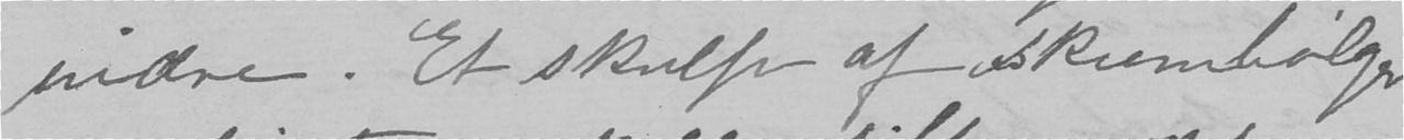indre. Et skvulp af skumbølger
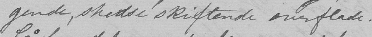gende, stedse skiftende overflade.
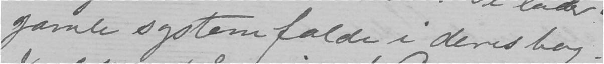gamle system falde i deres bog.
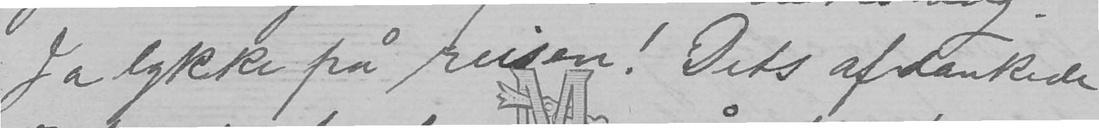Ja lykke på reisen! Dets afdankede
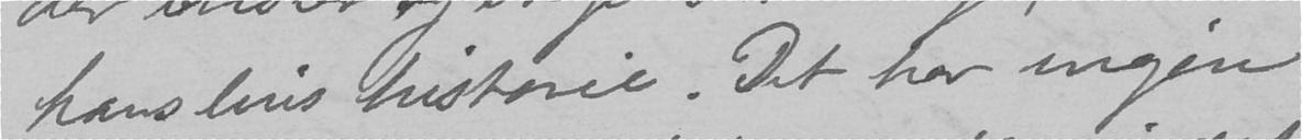hans livs historie. Det har ingen
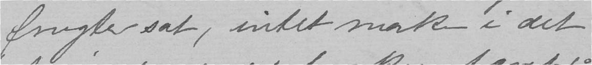frugter sat, intet mærke i det
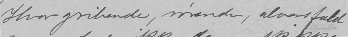Hvor gribende rørende, alvorsfuld
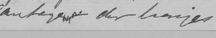antage der bringes
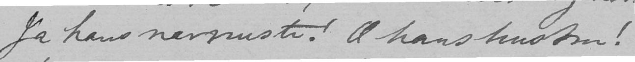Ja hans nærmeste! Og hans hustru!
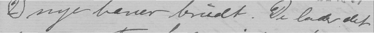nye baner brudt. De lader det
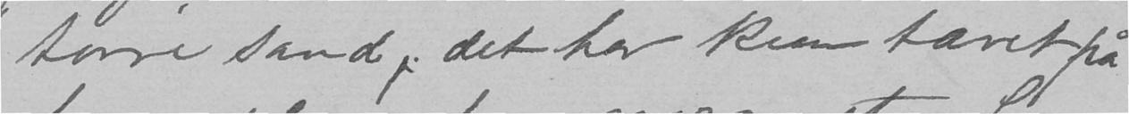tørre sand, det har kun tæret på
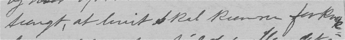tungt, at livet skal kunne forskus-
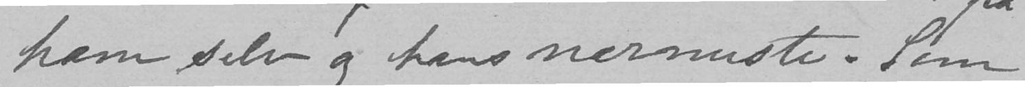ham selv og hans nærmeste. "Som
